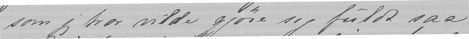som jeg tror vilde gjøre sig fuldt saa
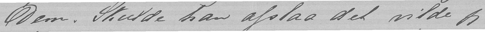Dem. Skulde han afslaa det vilde jeg
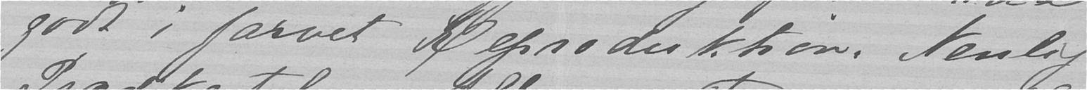godt i farvet Reproduktion. Nemlig
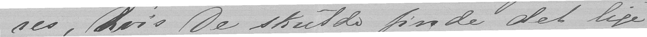res, hvis De skulde finde det lige-
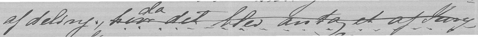afdeling, hvor det blev antaget af Jury-
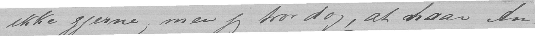ikke gjerne; men jeg tror dog, at naar An-
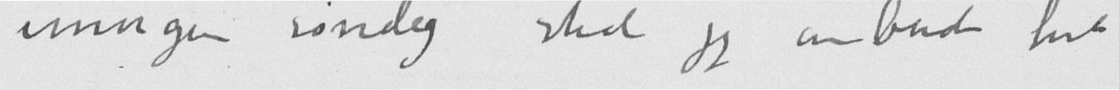imorgen søndag skal jeg arbeide hele
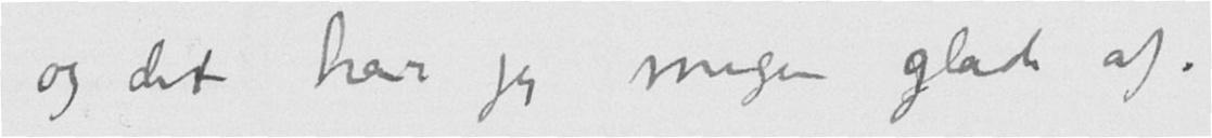og det har jeg megen glæde af.
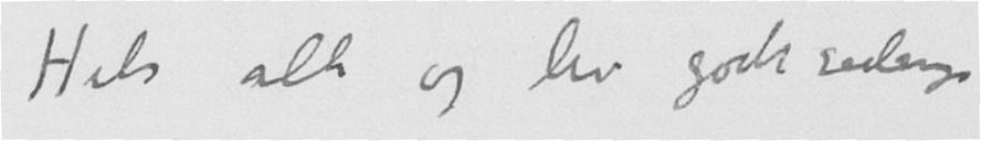Hils alle og lev godt saalenge
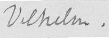Vilhelm.
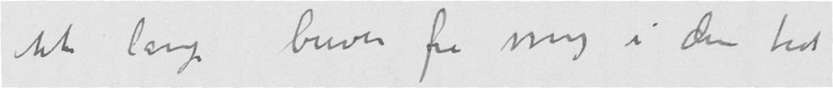ikke lange brever fra mig i den tid
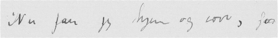Nu faar jeg hjem og sove, for
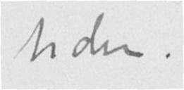tiden.
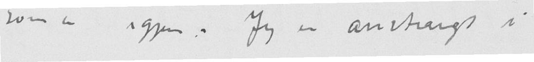som er igjen. Jeg er anstrængt i
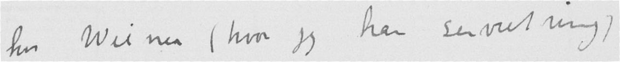hos Wiener (hvor jeg har servit mig)
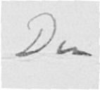Din
